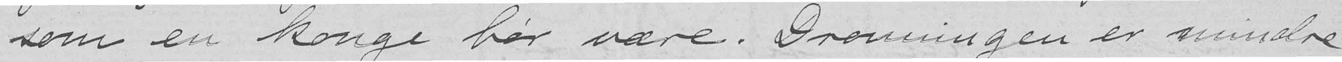som en konge bør være. Dronningen er mindre
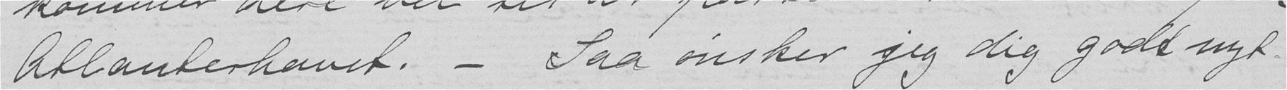Atlanterhavet. – Saa ønsker jeg dig godt nyt-
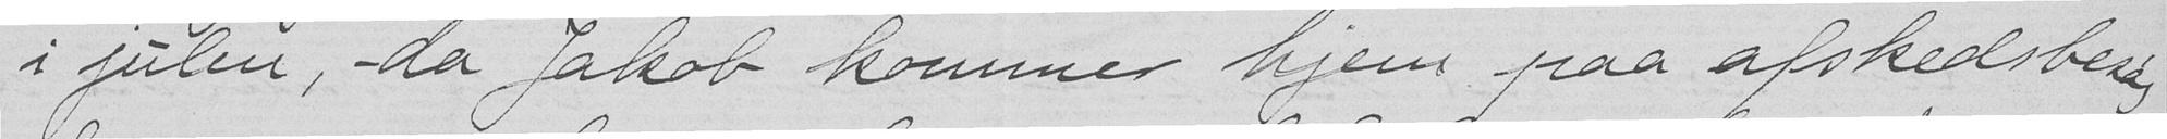i julen, – da Jakob kommer hjem paa afskedsbesøg
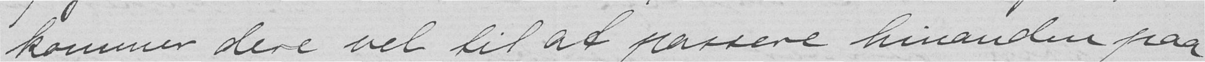kommer dere vel til at passere hinanden paa
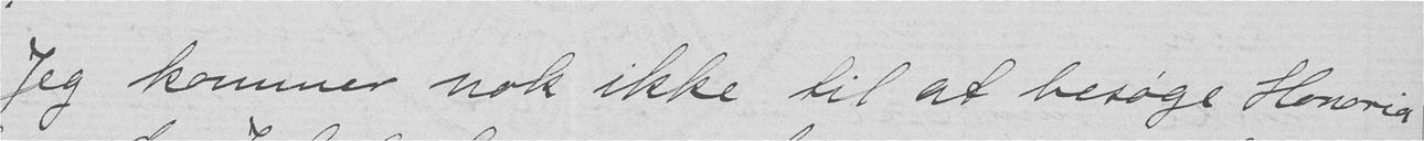Jeg kommer nok ikke til at besøge Honoria
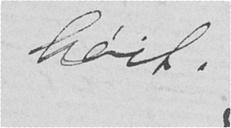høit.
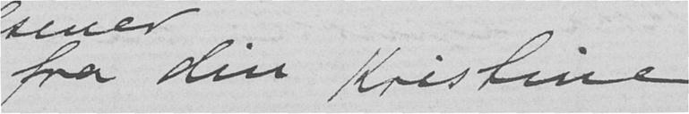fra din Kristine
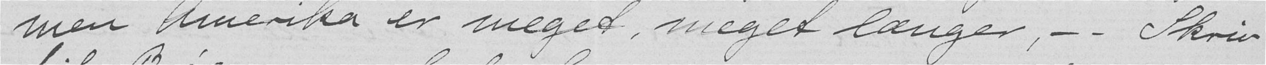men Amerika er meget, meget længer, – – Skriv
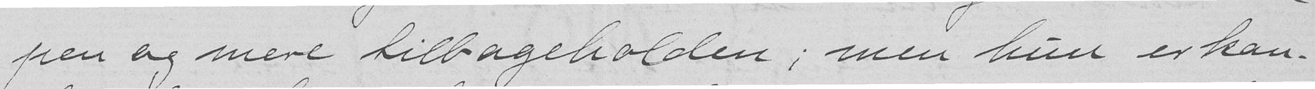pen og mere tilbageholden; men hun er kan-
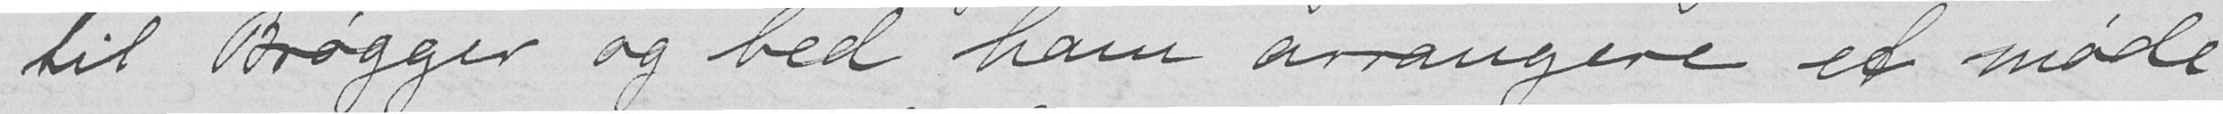til Brøgger og bed ham arrangere et møde
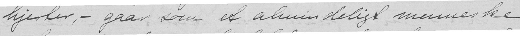hjerter, – gaar som et almindeligt menneske
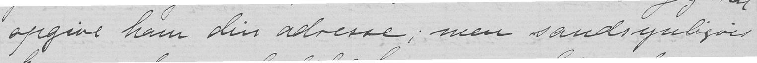opgive ham din adresse; men sandsynligvis
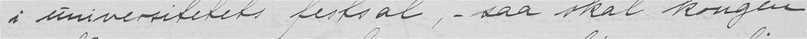i universitetets festsal, – saa skal kongen
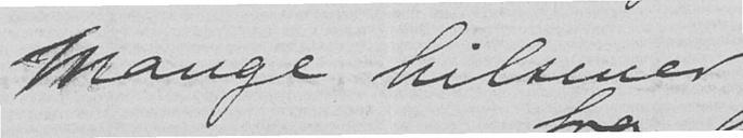Mange hilsener
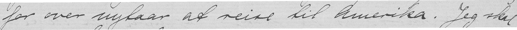for over nytaar at reise til Amerika. Jeg skal
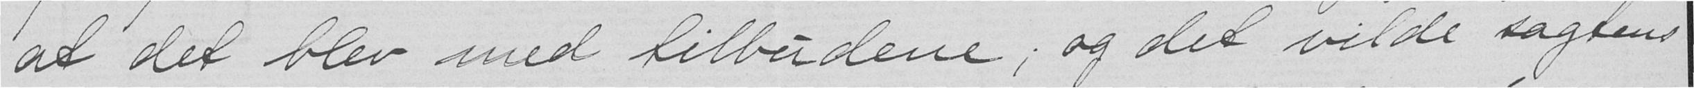at det blev med tilbudene; og det vilde sagtens
